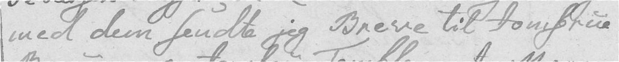med dem sendte jeg Breve til Jomfrue
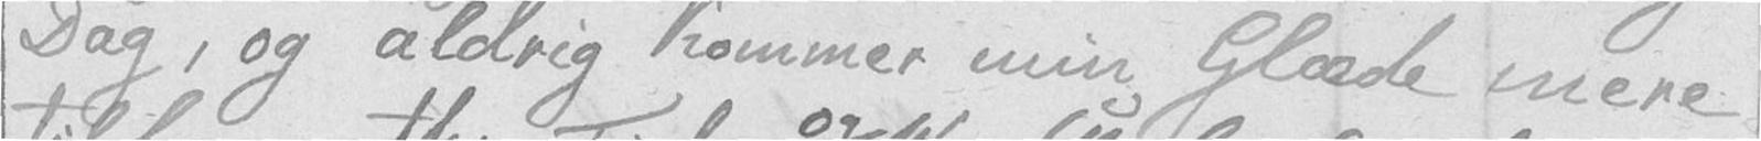Dag, og aldrig kommer min Glæde mere
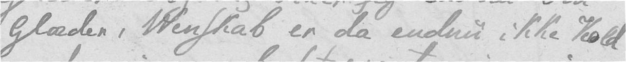Glæder, Venskab er da endnu ikke Kold
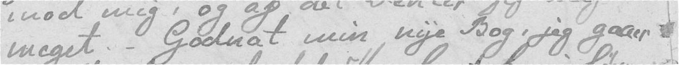meget. – Godnat min nye Bog, jeg gaaer
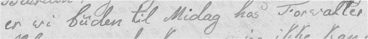er vi buden til Midag hos Forvalter
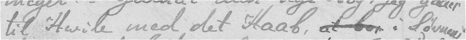til Hvile med det Haab, at bor i Søvnens
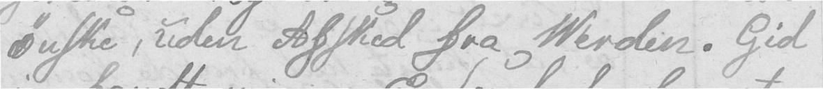ønske, uden Afsked fra Verden. Gid
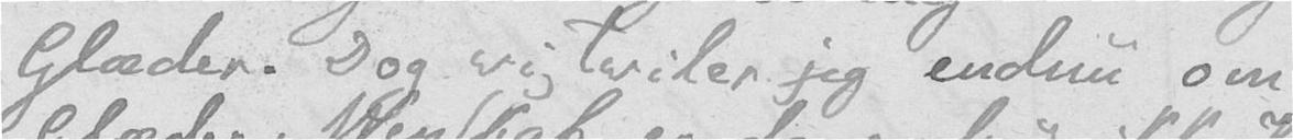Glæder. Dog vi tviler jeg endnu om
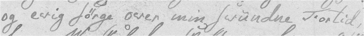og evig sørge over min svundne Fortid,
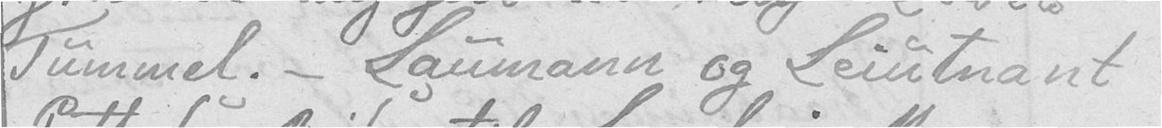Tummel. – Laumann og Leiutnant
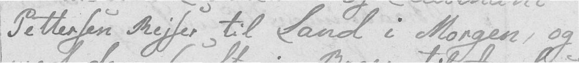Pettersen Rejser til Land i Morgen, og
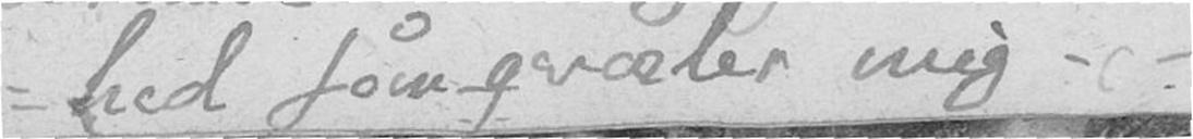-hed som qvæler mig – –
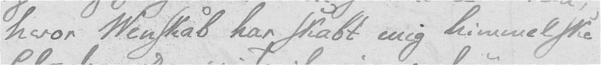hvor Venskab har skabt mig himmelske
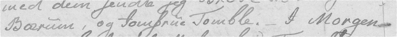Bærum, og Jomfrue Tomble. – I Morgen
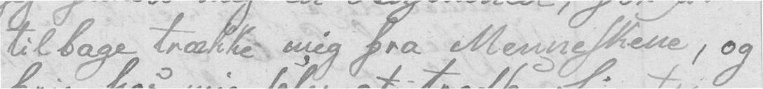tilbage trække mig fra Menneskene, og
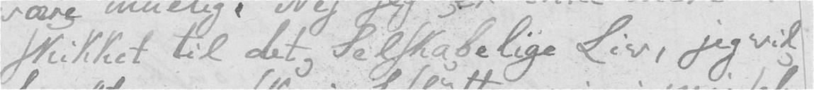skikket til det Selskabelige Liv, jeg vil
